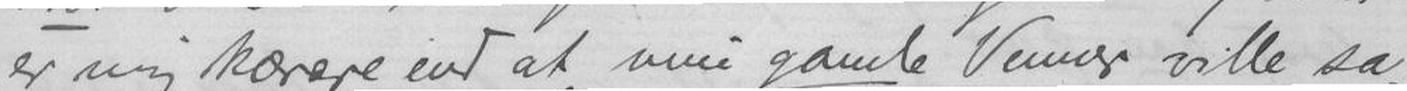er mig kærere end at mine gamle Venner ville sa-
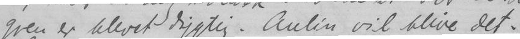gren er blevet dygtig. Aulin vil blive det.
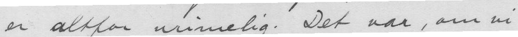er altfor urimelig. Det var, om vi
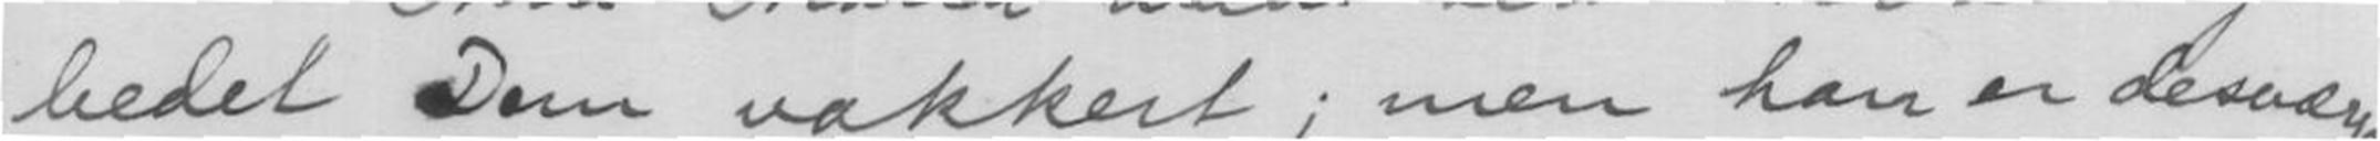bedet Dem vakkert; men han er desværre
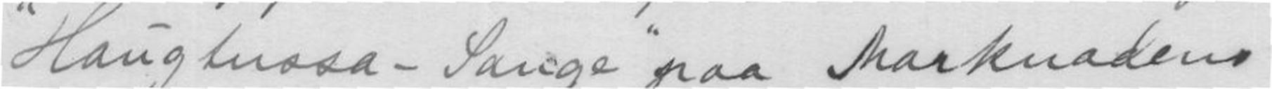"Haugtussa-Sange" paa Marknadens
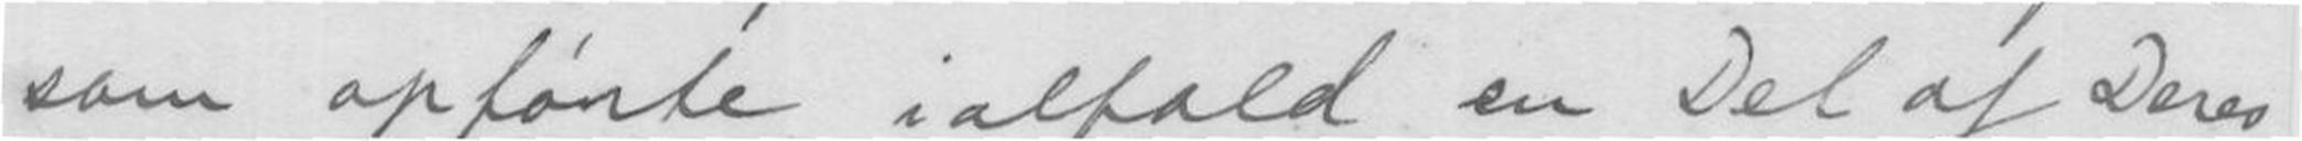som opførte ialfald en Del af Deres
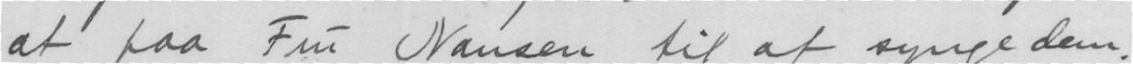at faa Fru Nansen til at synge dem.
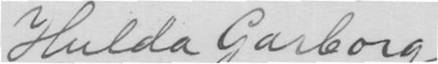Hulda Garborg
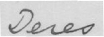Deres
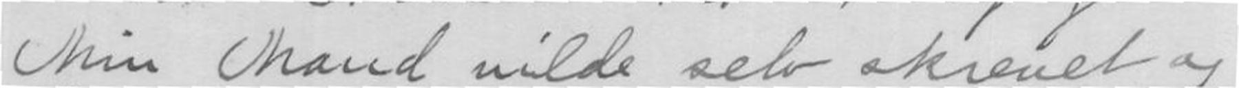Min Mand vilde selv skrevet og
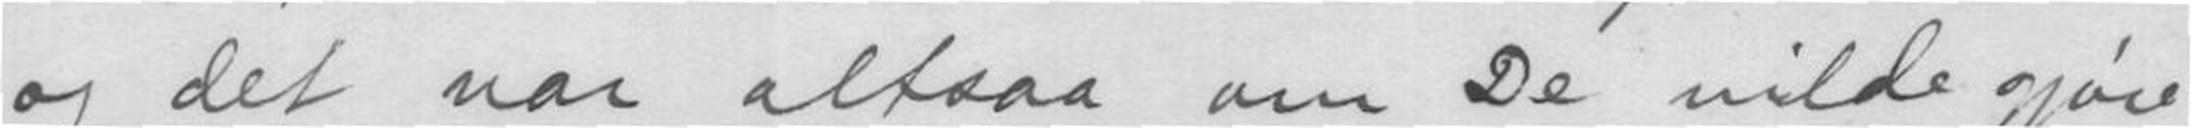og det var altsaa om De vilde gjøre
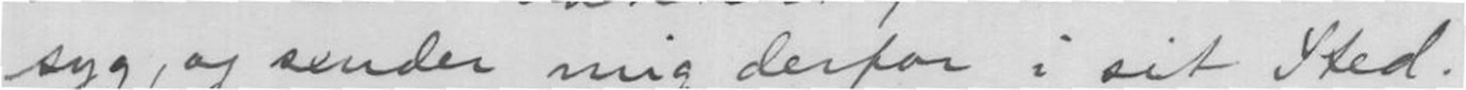syg, og sender mig derfor i sit Sted.
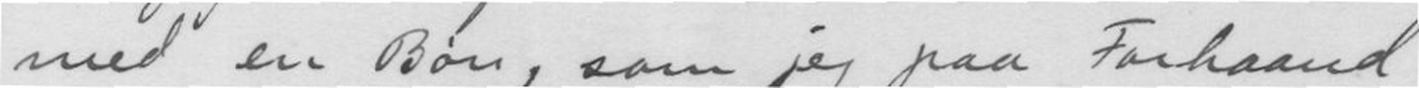med en Bøn, som jeg paa Forhaand
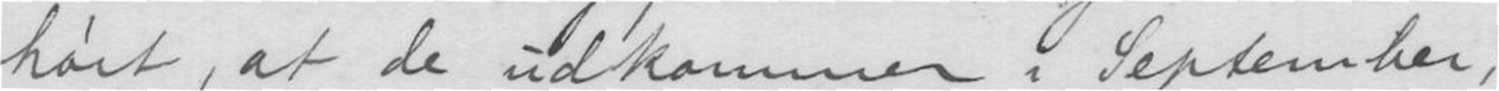hørt, at de udkommer i September;
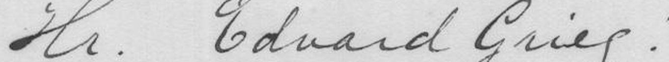Hr. Edvard Grieg!
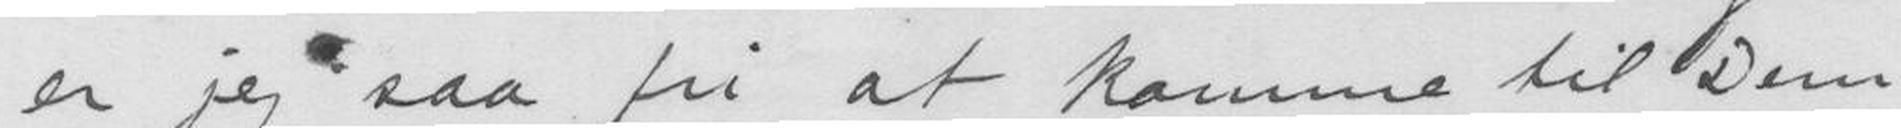er jeg saa fri at komme til Dem
